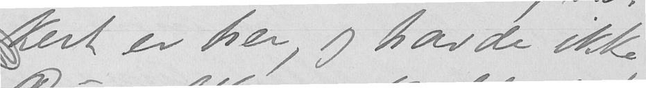kert er her, og havde ikke
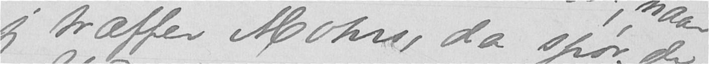jeg træffer Mohrs, da spør de
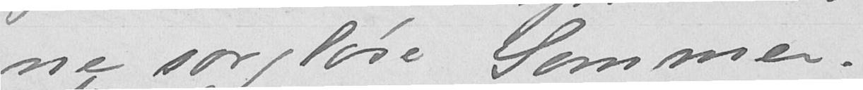ne sorgløse Sommer.
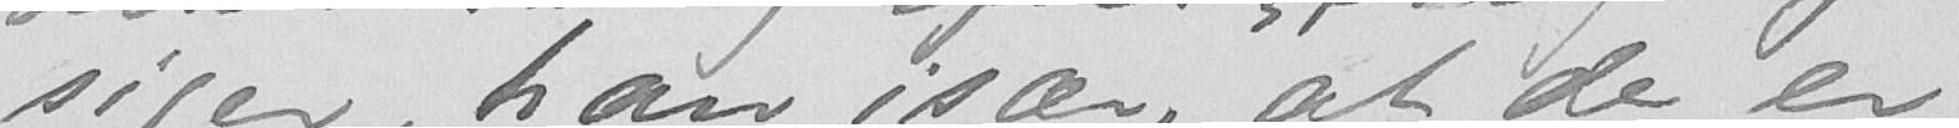siger, han især, at de er
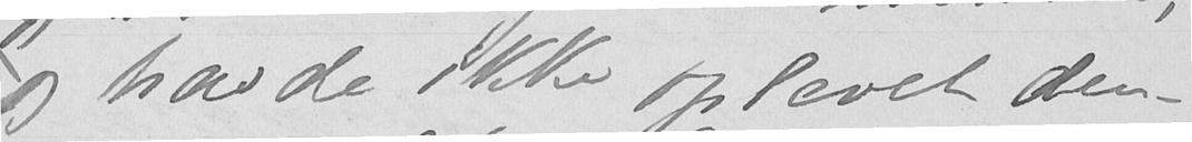og havde ikke oplevet den-
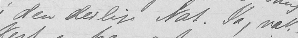i den deilige Nat. Ja, vak-
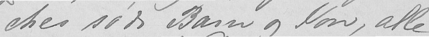ches søde Barn og Jon, alle-
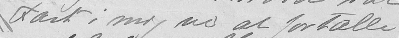Fart i mig ved at fortælle
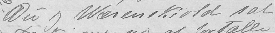Du og Werenskiold sat
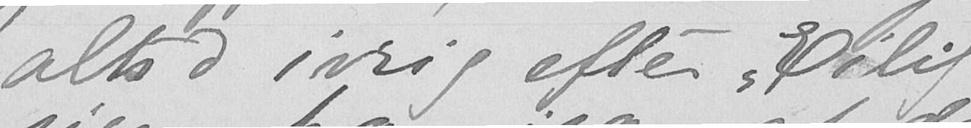altid ivrig efter "Eilif" og
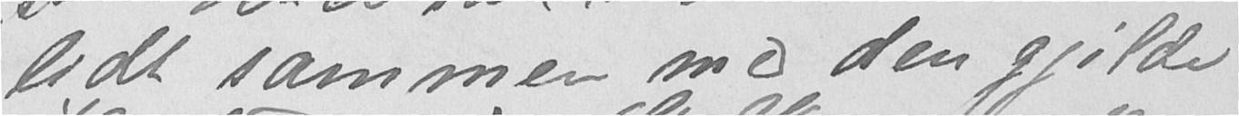lidt sammen med den gjilde
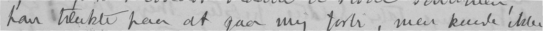han tænkte paa at gaa mig forbi, men kunde ikke
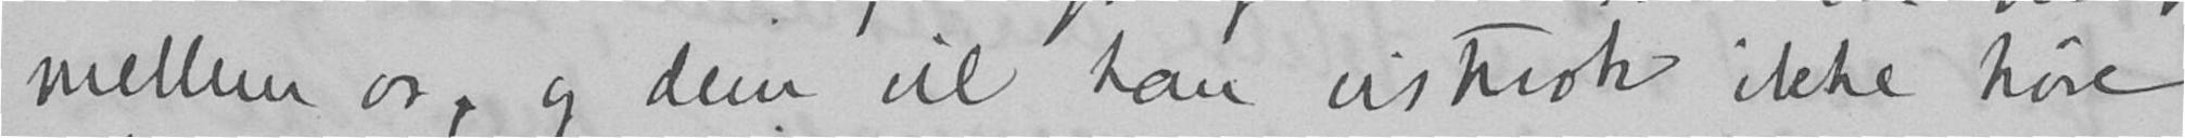mellem os, og dem vil han vistnok ikke høre.
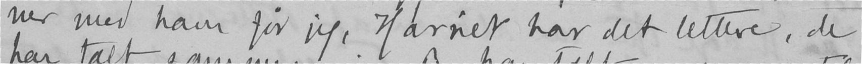ner med ham før jeg, Harriet har det lettere, de
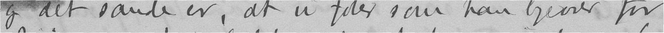og det sande er, at vi føler som han ligeover for
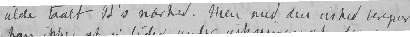vilde taalt B's nærhed. Men med den vished beregner
